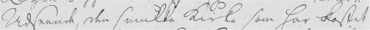Udseende, den smukke Kirke som har kostet
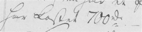har kostet 700 rd. -
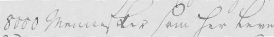8000 Mennesker som her leve
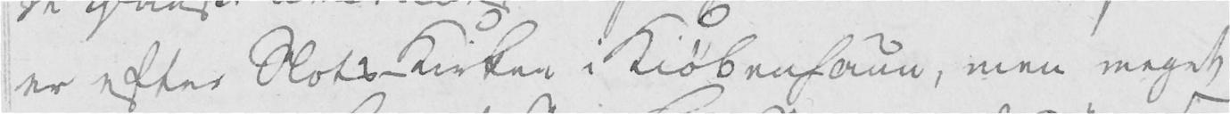er efter Slots-Kirken i Kiøbenhaun, men meget
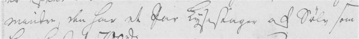mindre, den har et Par Lysestager af Sølv som
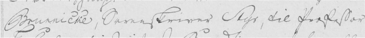Brunnicke, Sorenskriver Styr, til Professor
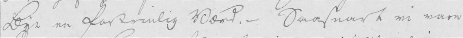Bye en fortrinlig Værd. - Saasnart vi vare
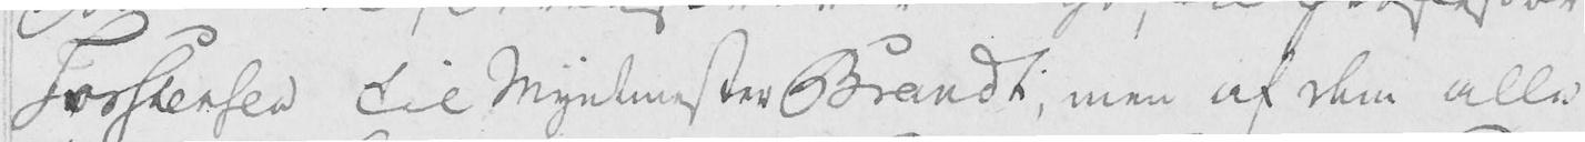Torstensen til Myntmester Brandt; men af dem alle
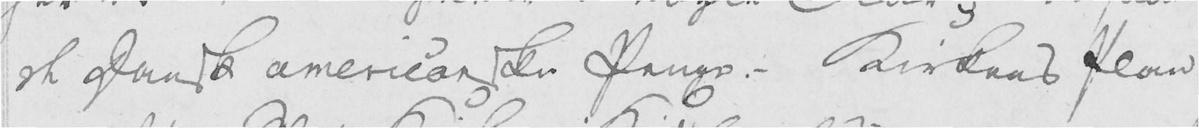de Dansk americanske Penge. - Kirkens Plan
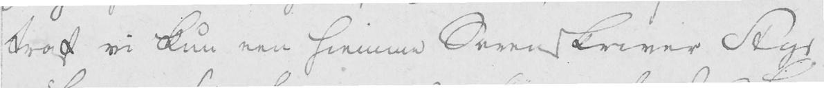traf vi kun een hiemme Sorenskriver Styr
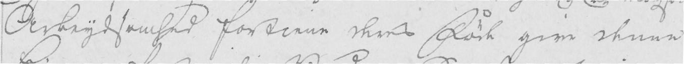Arbeydsomhed fortiene deres Føde give denne
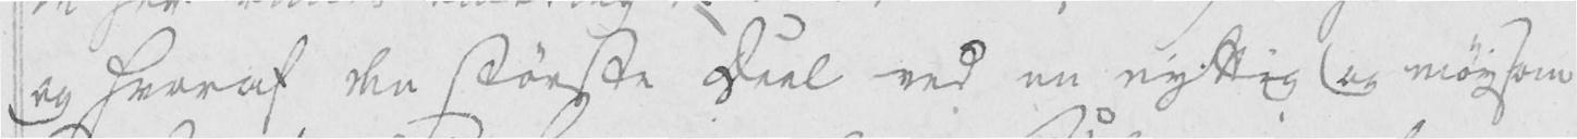og hvoraf den største Deel ved en nyttig og møysom
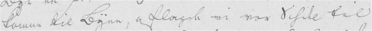komne til Byen, aflagde vi vor Visite til
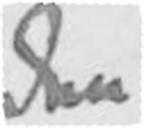de
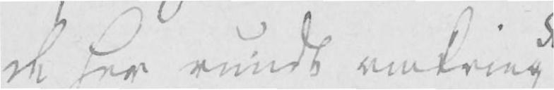de her rundt omkring
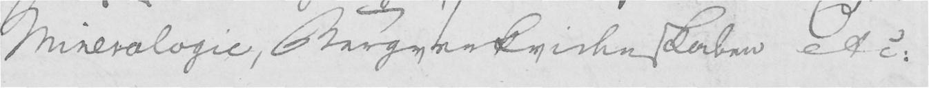Mineralogie, Bergverkvidenskaben etc.
